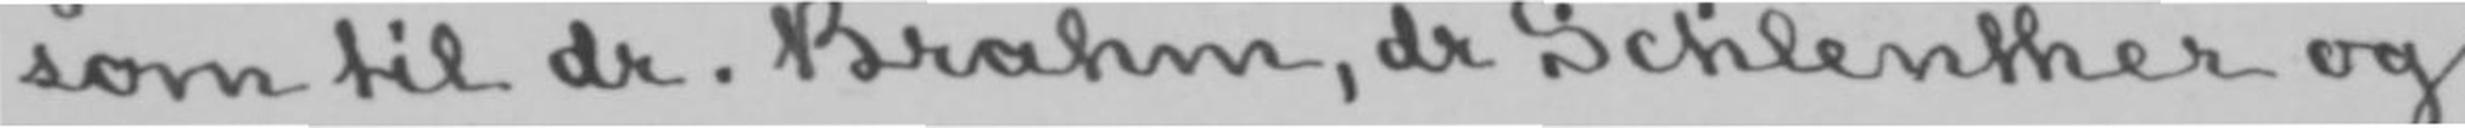som til dr. Brahm, dr Schlenther og
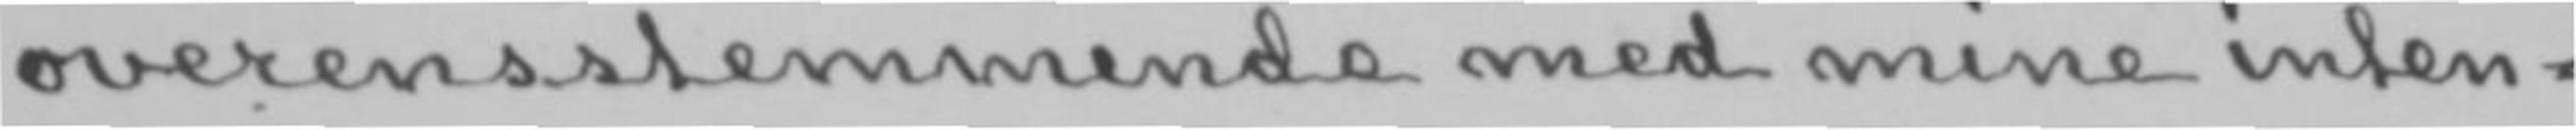overensstemmende med mine inten-
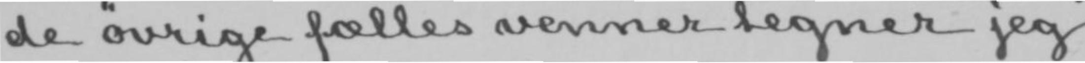de øvrige fælles venner tegner jeg
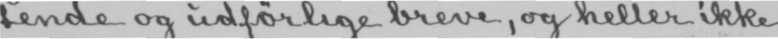tende og udførlige breve, og heller ikke
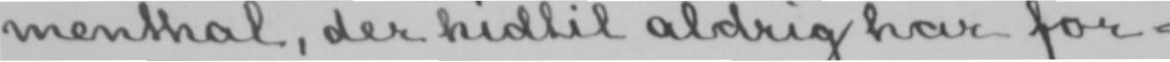menthal, der hidtil aldrig har for-
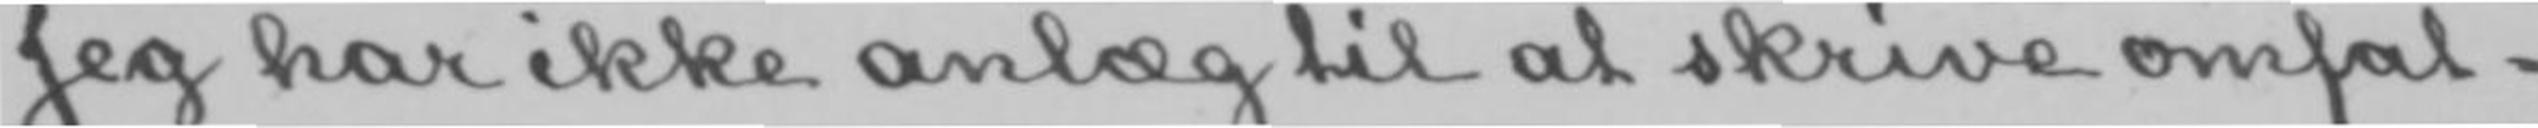Jeg har ikke anlæg til at skrive omfat-
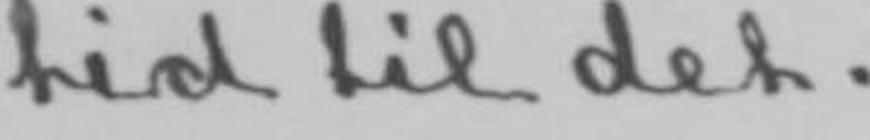tid til det.
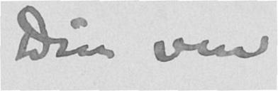Din venn
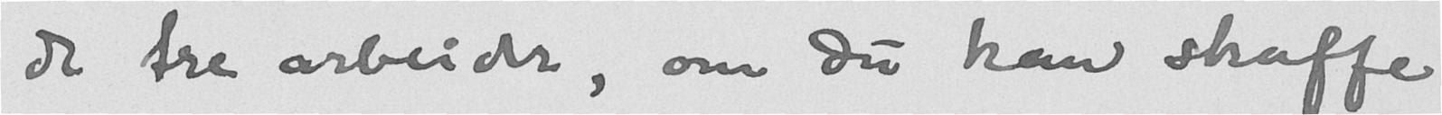de tre arbeider, om du kan skaffe
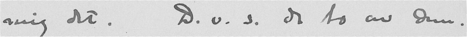mig det. D.v.s. de to av dem.
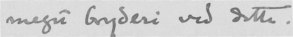meget bryderi ved dette.
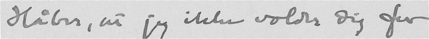Håber, at jeg ikke volder dig for
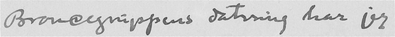Broncegruppens datering har jeg.
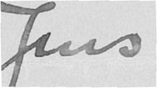Jens
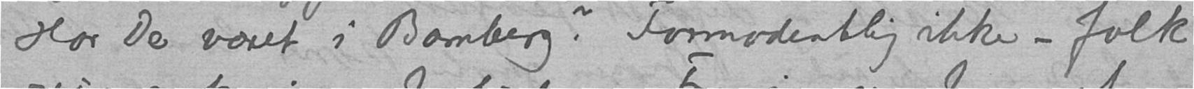Har De været i Bamberg? Formodentlig ikke – folk
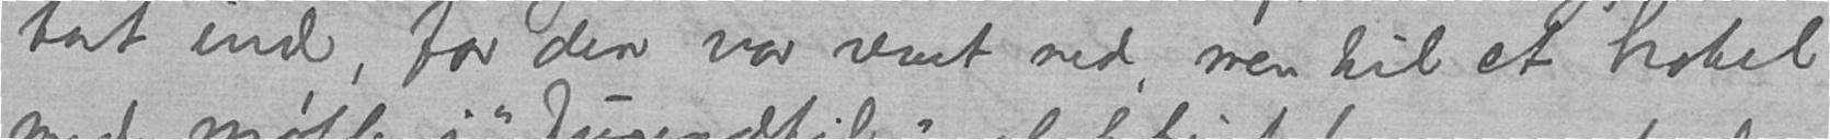tat ind, for den var revet ned, men til et hotel
